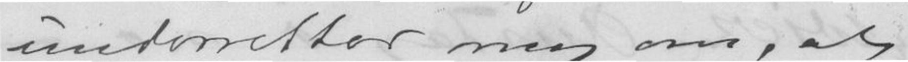underretter mig om, at
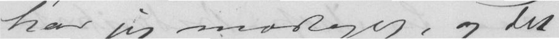har jeg modtaget, og det
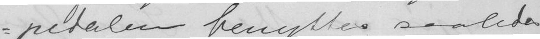pedalen benyttes saaledes
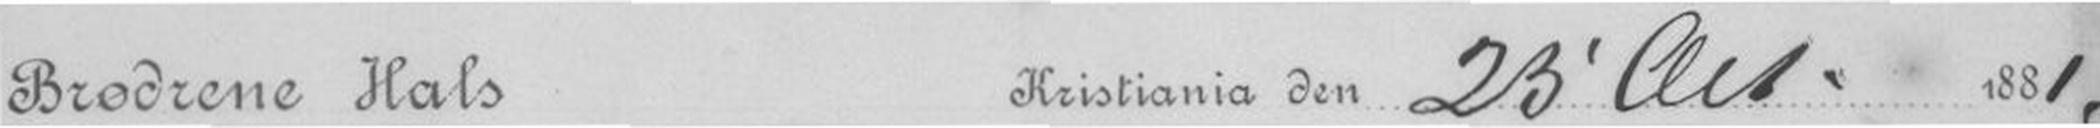Brødrene Hals Kristiania den 25 Oct. 1881.
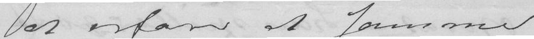at erfare at samme
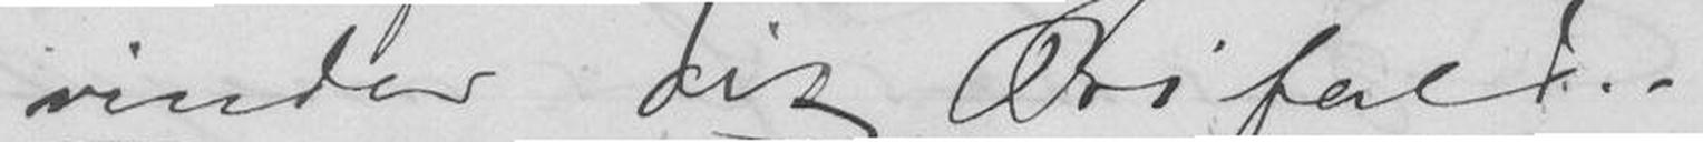vinder dit Bifald.
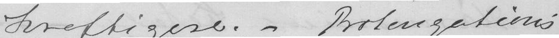kraftigere. - Bolengations-
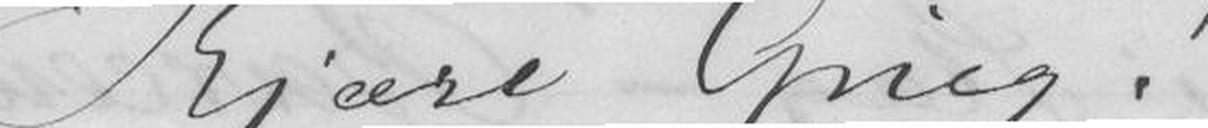Kjære Grieg!
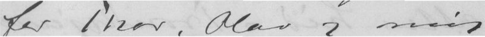for Thor, Olav og mig
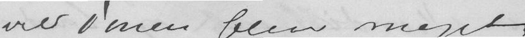vil Tonen blive meget
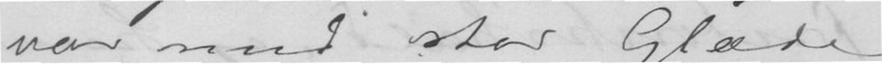var med stor Glæde
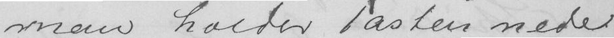man holder Tasten nede
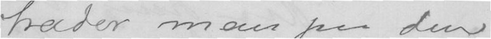træder man paa den
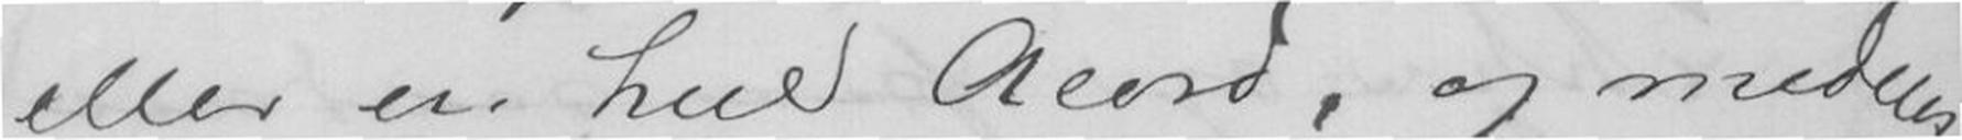eller en heel Acord, og medens
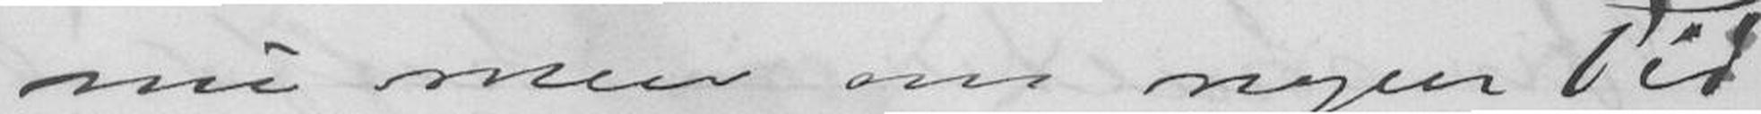nu men om nogen Tid
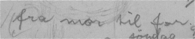fra mor til far.
